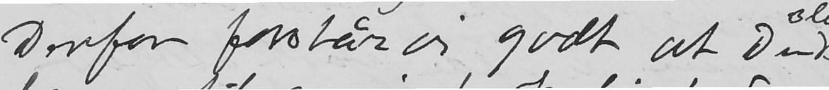Derfor forstår jeg godt at Du
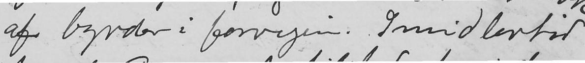af byrder i forvejen. Imidlertid
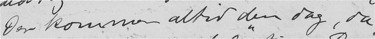Der kommer altid den dag, da
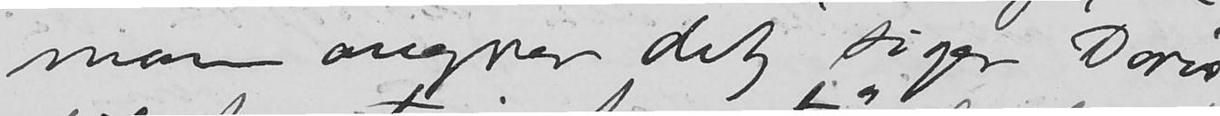man angrer det," siger Doris
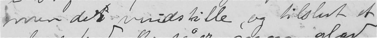mer det vindstille, og tilslut et
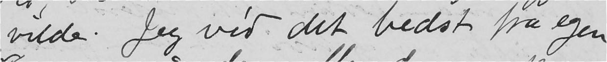vilde. Jeg véd det bedst fra egen
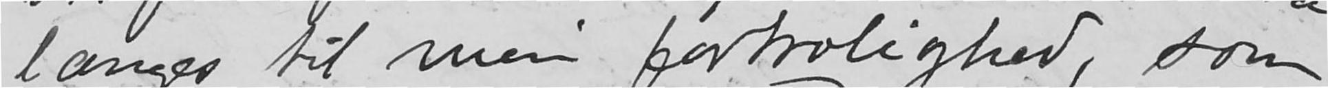længes til min fortrolighed, som
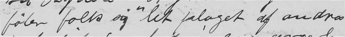føler folk sig let plaget af andres
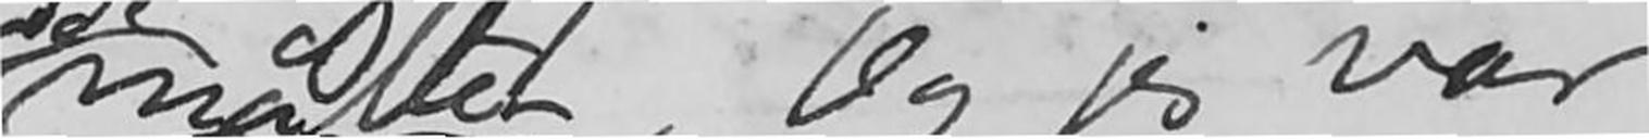måttet. Og jeg var
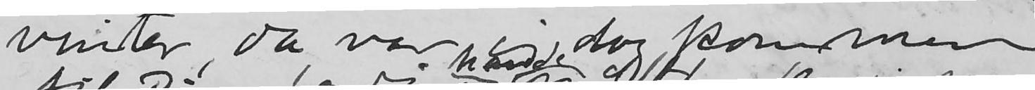vinter, da var jeg dog kommen
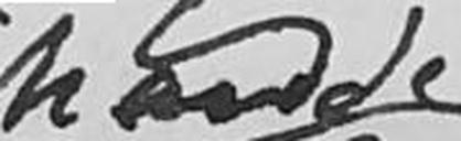havde
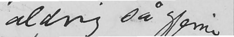aldrig så gjerne
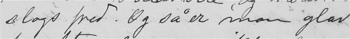slags fred. Og så er man glad
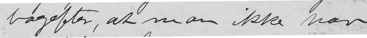bagefter, at man ikke har
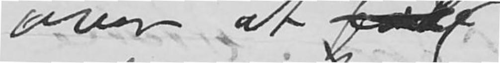over at
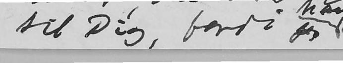til Dig, fordi jeg
